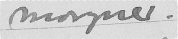morgener!
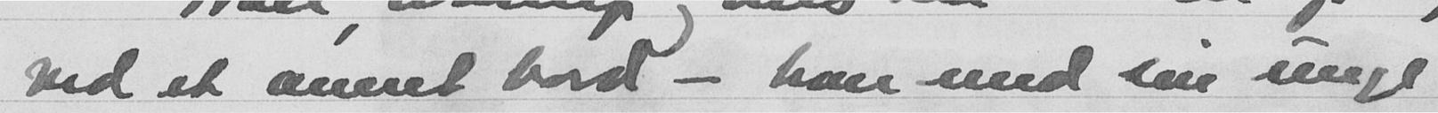ved et annet bord - han med sin unge
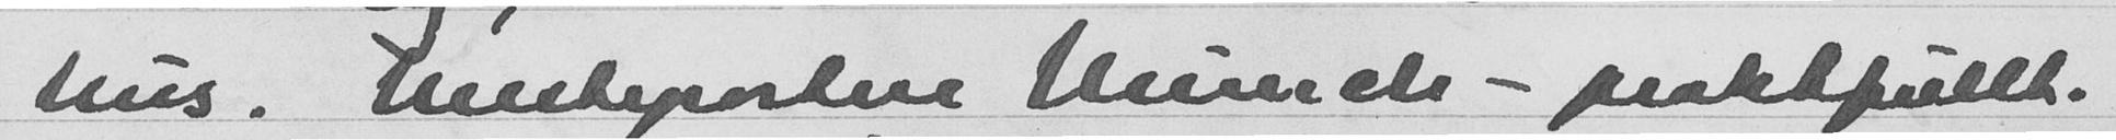hus. Mite posten Munch - praktpullt.
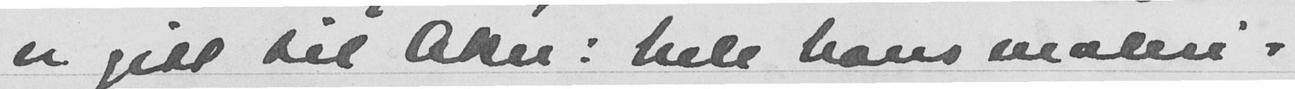er gitt til Aker: hele hans maleri-
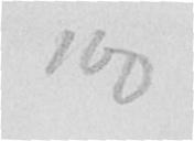100
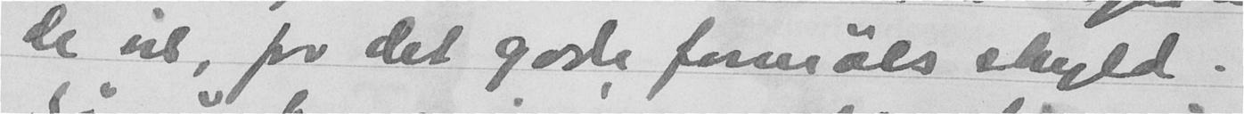de vil, for det gode formåls skyld.
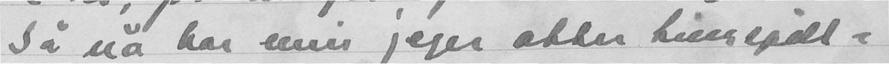Så nå har min jeger atter linespill-
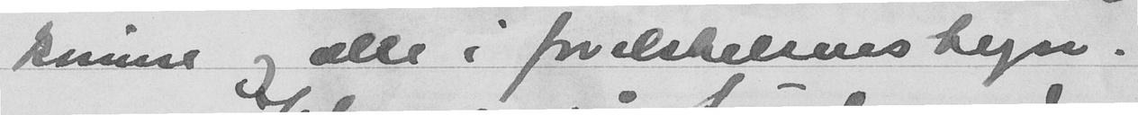kone og alle i forelskelsens tegn.
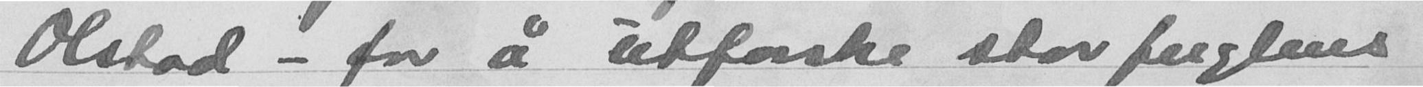Olstad - for å utforske storfuglens
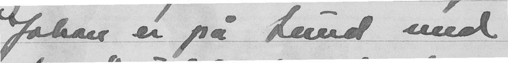Johan er på Lund med
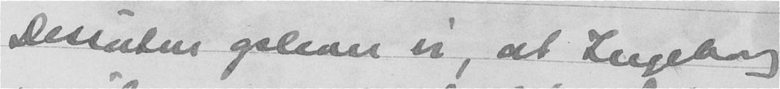Dessuten oplever vi, at Ingeborg
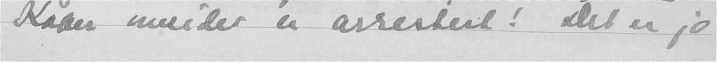Køber omsider er arrestert! Det er jo
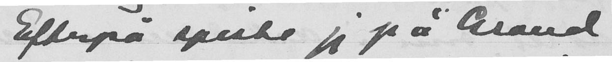Efterpå spiste jeg på Grand
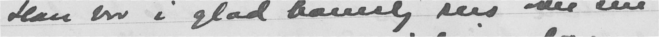Han var i glad barnslig rus over sin
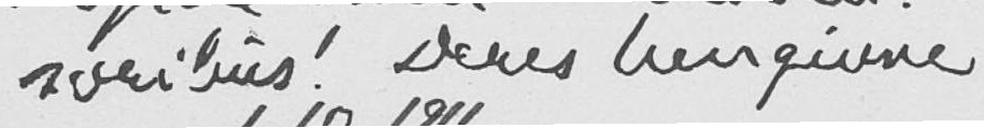soribus! Deres hengivne
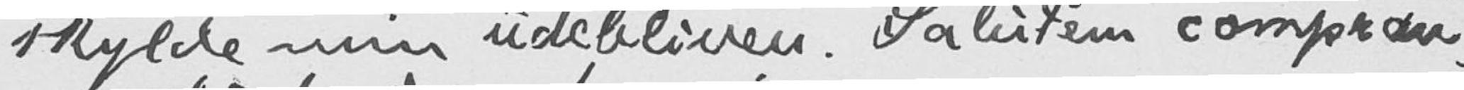skylde min udebliven. Salutem compran-
